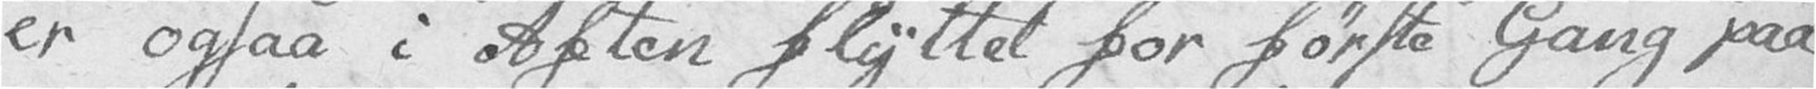er ogsaa i Aften flyttet for første Gang paa
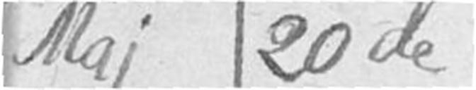Maj 20de
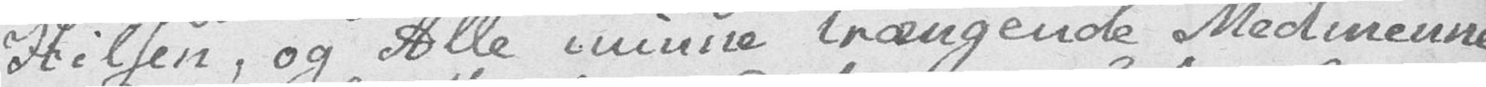Hilsen, og Alle minne trængende Medmenne
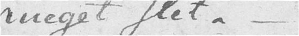meget slet. –
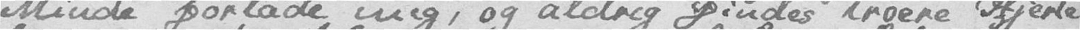Minde forlade mig, og aldrig findes troere Hjerte
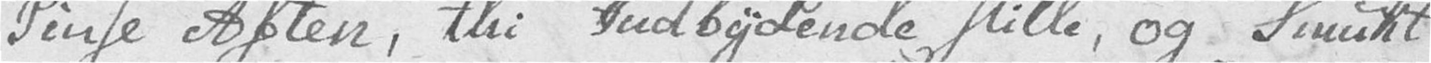Pinse Aften, thi Indbydende stille, og Smukt
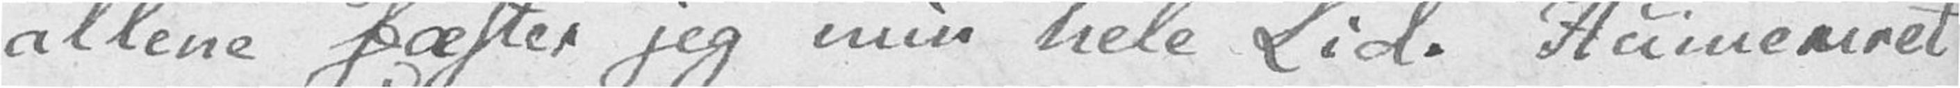allene fæster jeg min hele Lid. Humeuret
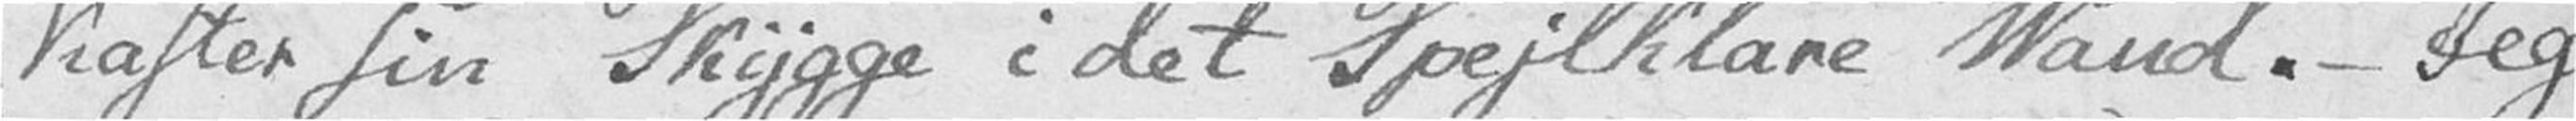kaster sin Skygge i det Spejlklare Vand. – Jeg
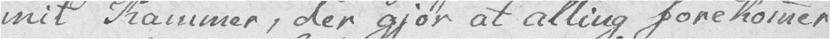mit Kammer, der gjør at alting forekommer
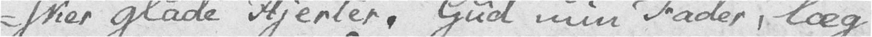-sker glade Hjerter. Gud min Fader, læg
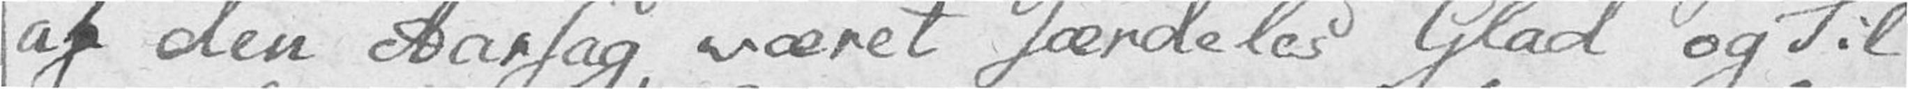af den Aarsag været særdeles Glad og Til
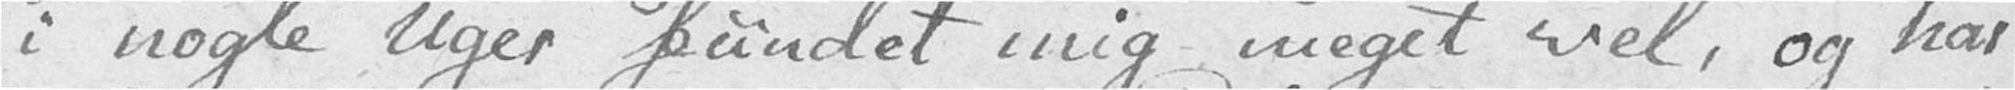i nogle Uger fundet mig meget vel, og har
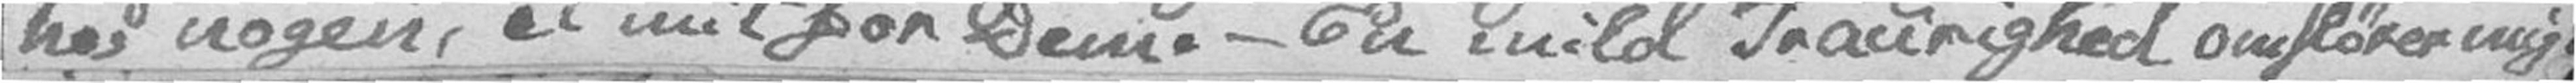hos nogen, et mit for Dem. – En mild Traurighed omslører mig.
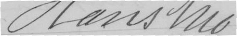Hans Mo
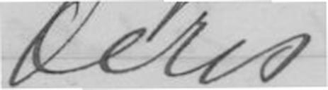Deres
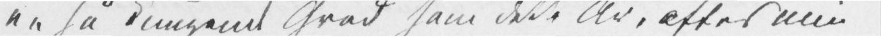en så knugende Grad som dette År, efter min
